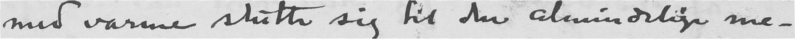med varme slutte sig til den "almindelige me-
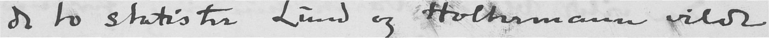de to statister Lund og Holtermann vilde
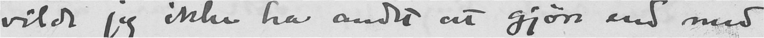vilde jeg ikke ha andet at gjøre end med
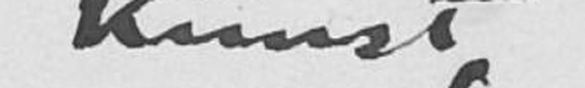kunst
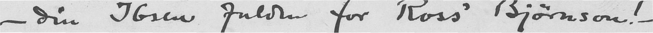- din Ibsen falden for Ross' Bjørnson! -
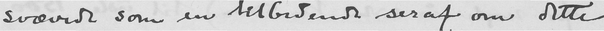svævede som en tilbedende seraf om dette
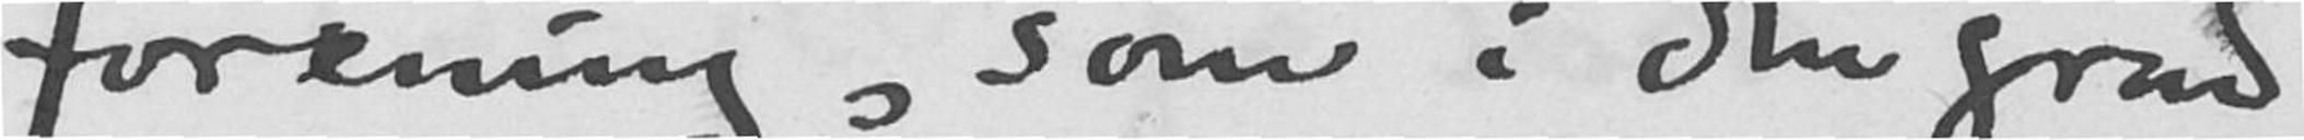forening, som i den grad
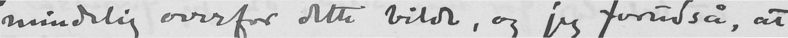mindelig overfor dette bilde, og jeg forudså, at
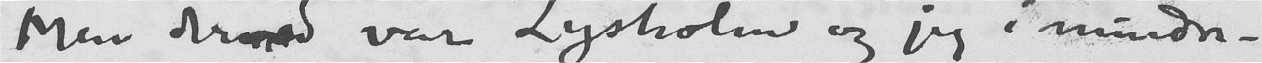Men derved var Lysholm og jeg i mindre-
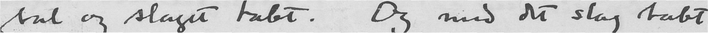tal og slaget tabt. Og med det slag tabt
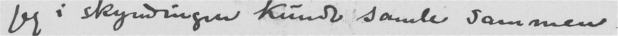jeg i skyndingen kunde samle sammen.
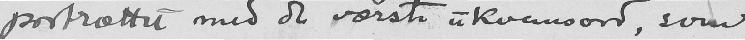portrættet med de værste ukvemsord, som
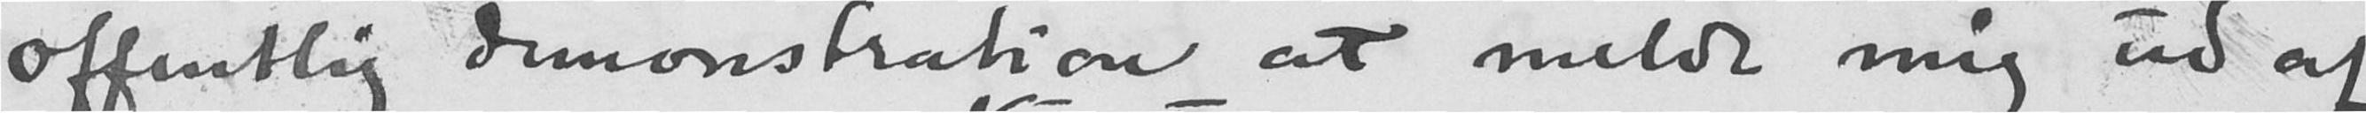offentlig demonstration at melde mig ud af
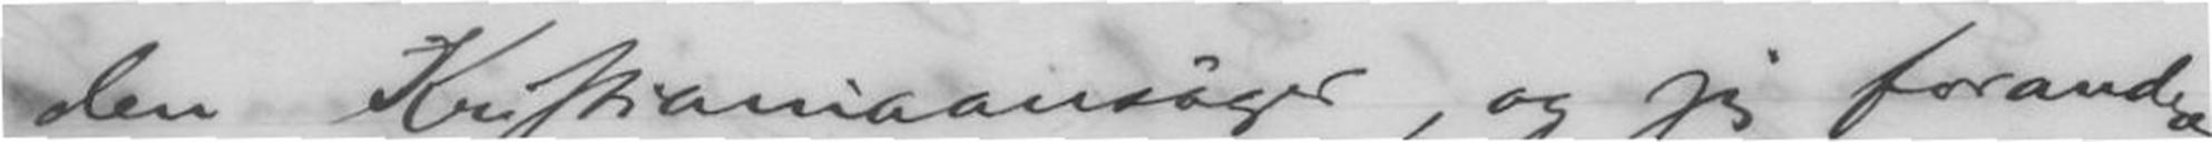den Kristianiaansøger, og jeg forandre-
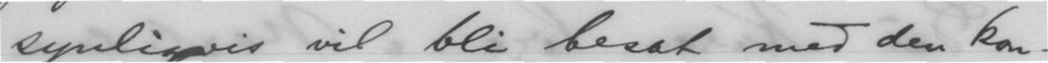synligvis vil bli besat med den kon-
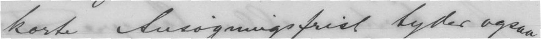korte Ansøgningsfrist tyder ogsaa
